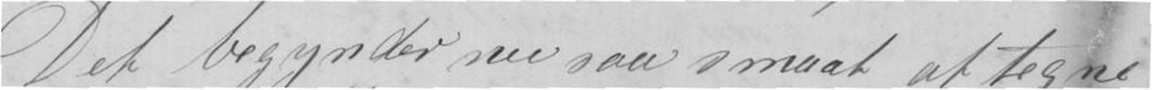Det begynder nu saa smaat at tegne
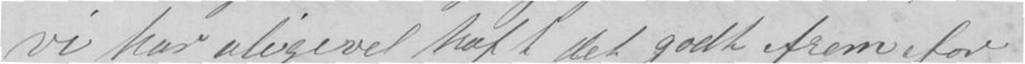vi har aligevel haft det godt fremfor
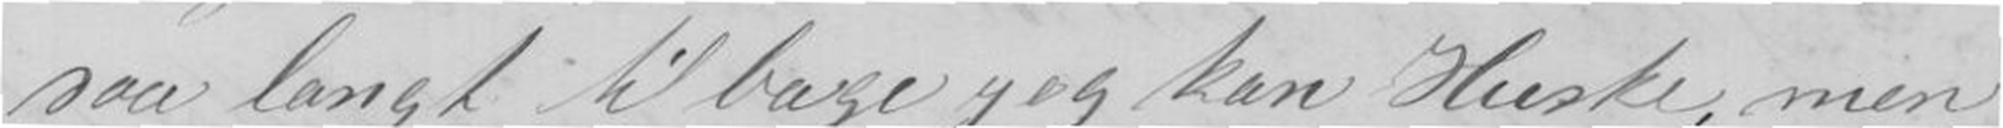saa langt tilbage jeg kan Huske, men
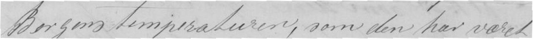Bergens temperaturen, som den har været
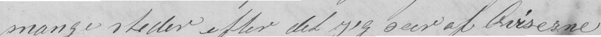mange steder efter det jeg seer af Aviserne.
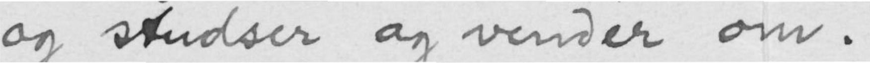og studser og vender om.
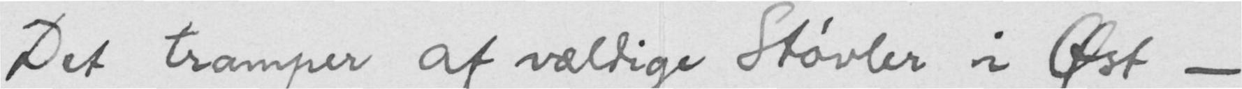Det tramper af vældige Støvler i Øst -
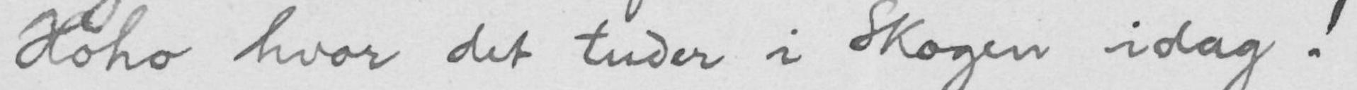Hoho hvor det tuder i Skogen idag!
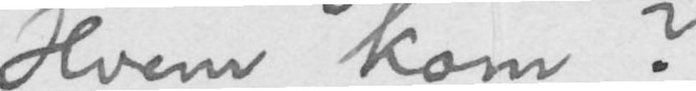Hvem kom?
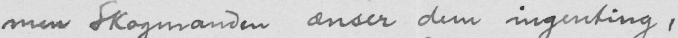men Skogmanden ænser dem ingenting,
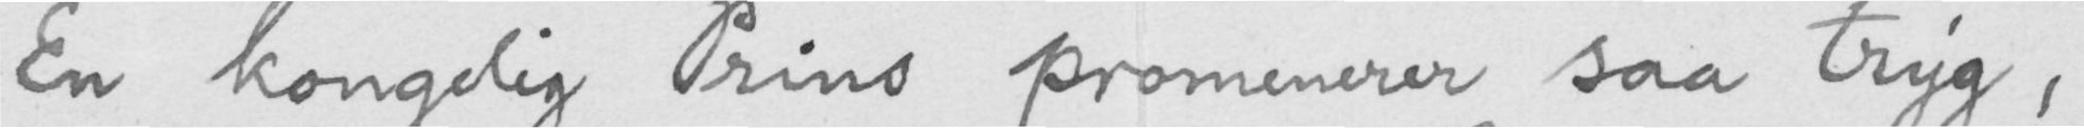En kongelig Prins promenerer saa tryg,
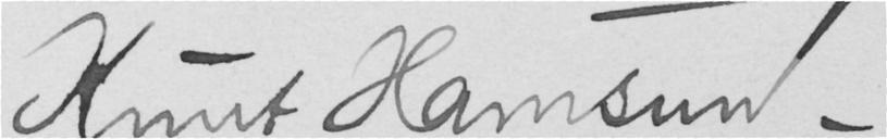Knut Hamsun.
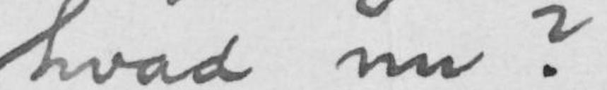hvad nu?
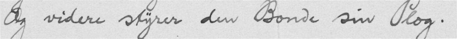Og videre styrer den Bonde sin Plog.
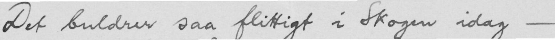Det buldrer saa flittigt i Skogen idag -
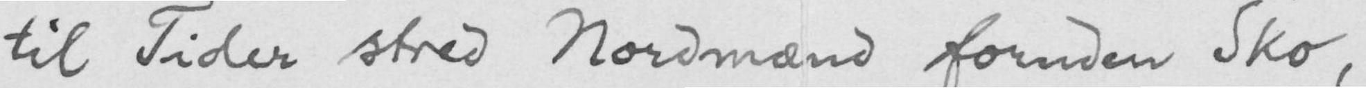til Tider stred Nordmænd foruden Sko,
